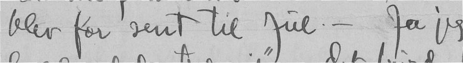blev for sent til Jul. - Ja jeg
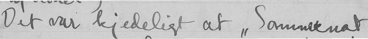Det var kjedeligt at "Sommernat
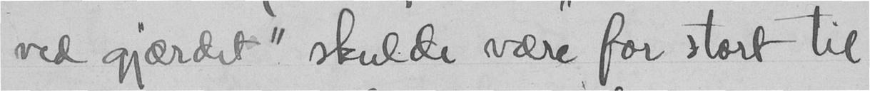ved gjærdet" skulde være for stort til
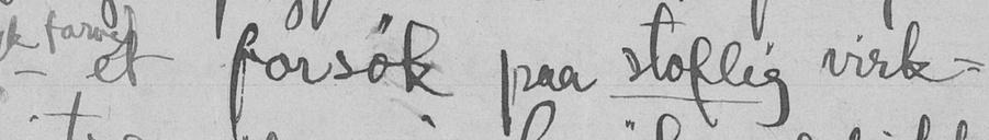- et forsøk paa stoflig virk-
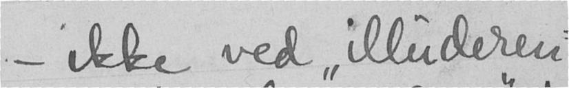- ikke ved "illuderen-
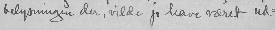belysningen der, vilde jo have været ud-
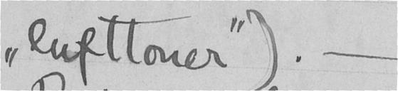"lufttoner"). -
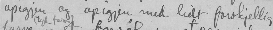opigjen og opigjen med lidt forskjellig
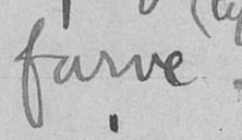farve
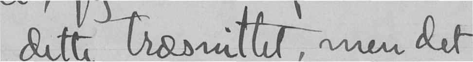dette træsnittet, men det
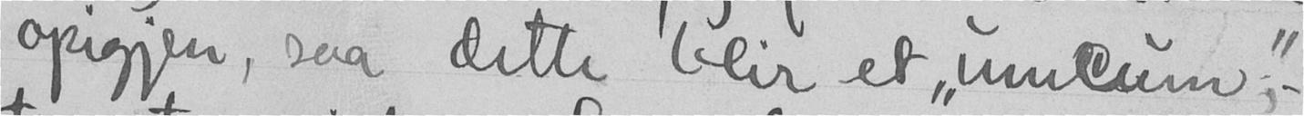opigjen, saa dette blir et "unicum"; -
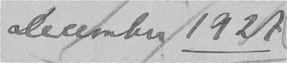december 1927
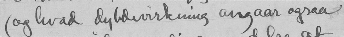(og hvad dybdevirkning angaar ogsaa
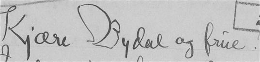Kjære Bydal og Frue.
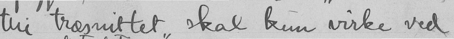thi træsnittet skal kun virke ved
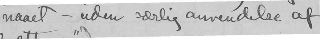naaet - uden særlig anvendelse af
